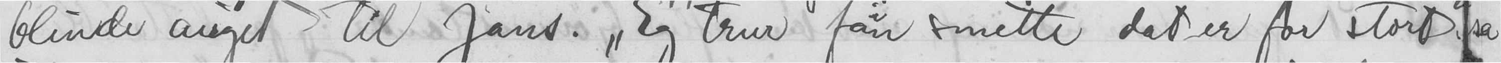blinde auget til Jans. "Eg trur fan smette dat er for stort", sa
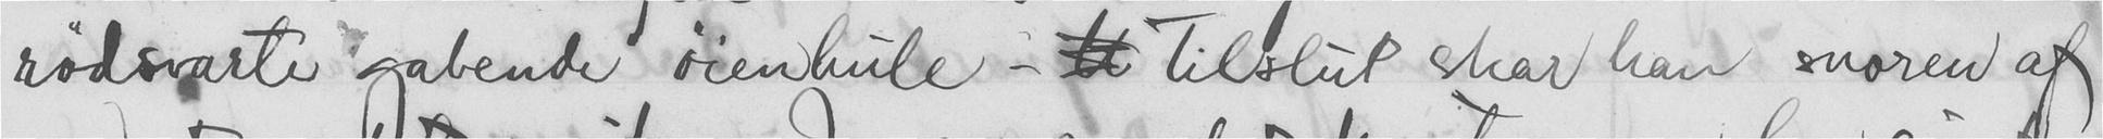rødsvarte gabende øienhule -  tilslut skar han snoren af
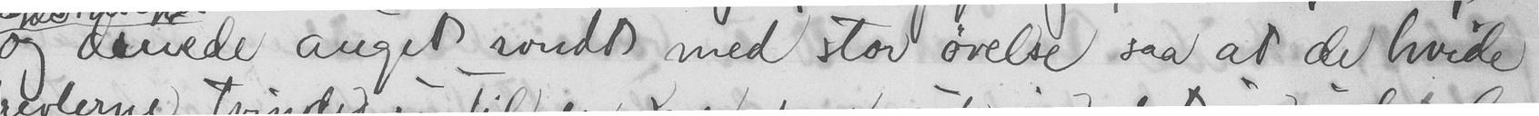og dreiede auget rondt med stor øvelse saa at de hvide
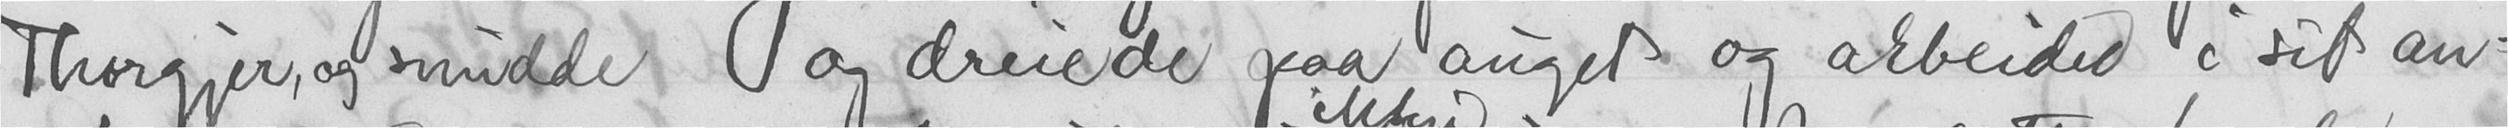Thorgjer, og snudde og dreiede paa auget og arbeided i sit an-
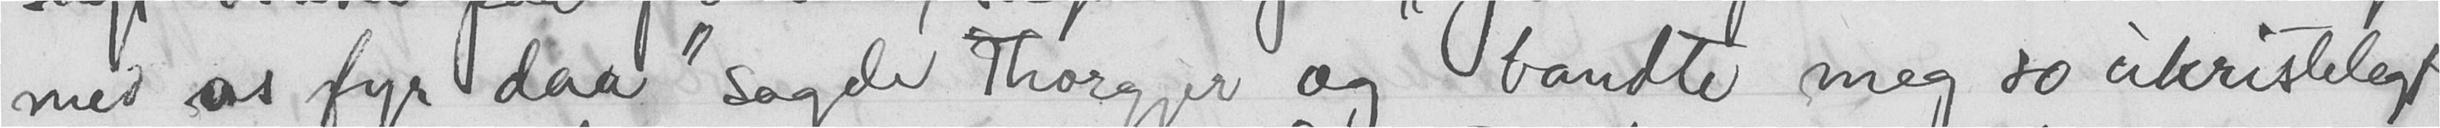med os fyr daa" sagde Thorgjer og bandte meg so ukristelegt
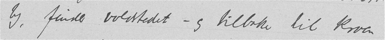by, finder voldstedet – og tilbake til kroen
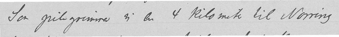Saa pilegrimmer vi en 4 kilometer til Norring
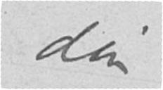din
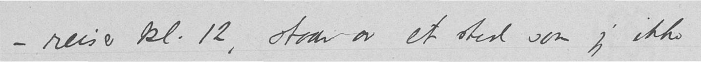– reiser kl. 12, staar av et sted som jeg ikke
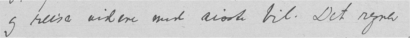og reiser videre med sisste bil. Det regner
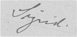Sigrid.
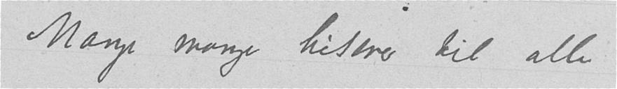Mange mange hilsener til alle
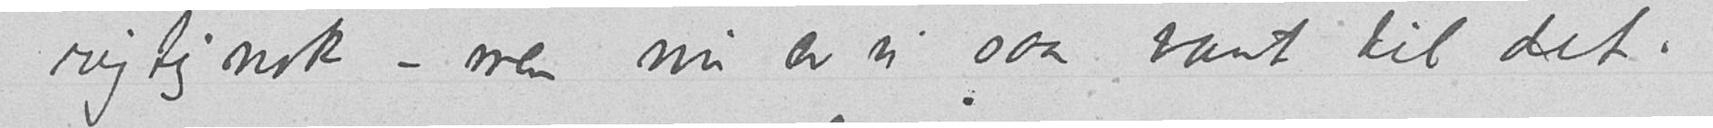riktignok – men nu er vi saa vant til det.
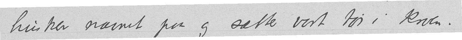husker navnet paa og sætter vort tøi i kroen.
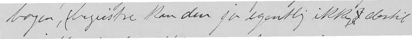bogen, (begeistre kan den jo egentlig ikke; dertil
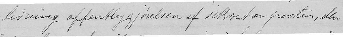ledning offentliggjørelsen af sekretærposten, eller
